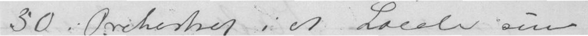50 i Orchestret i et Locale som
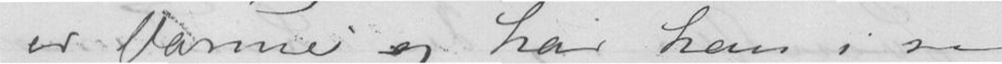er Varme og har han i sin
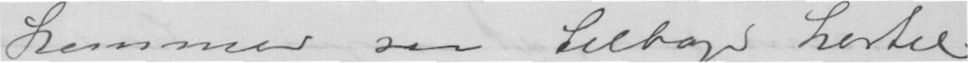kommer saa tilbage hertil
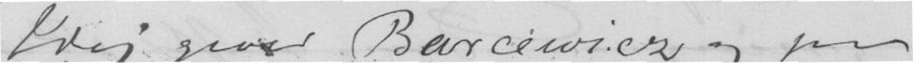Idag giver Barcewicz og paa
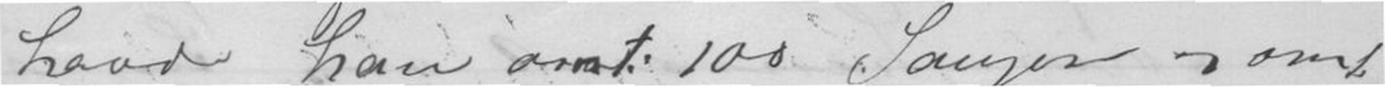havde han snart 100 Sanger og omt
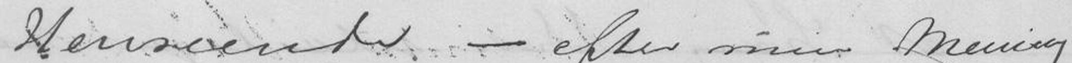Henseende - efter min Mening
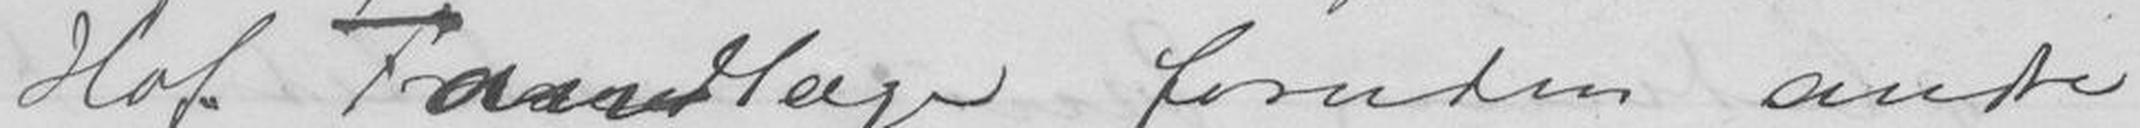Hof Tandlæge foruden andre
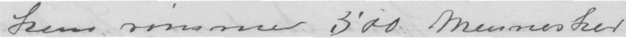kan romme 500 Mennesker
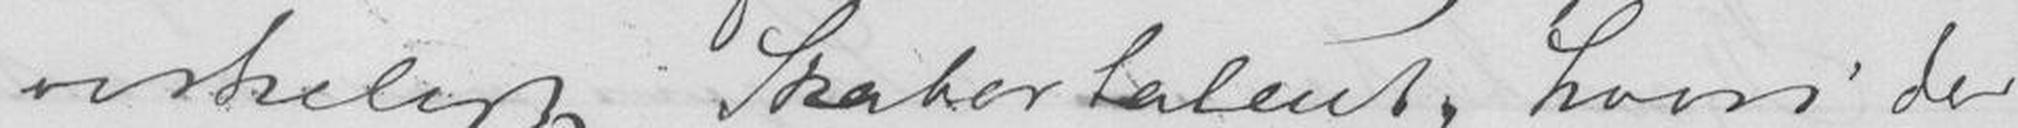virkelig Skabertalent, hvori der
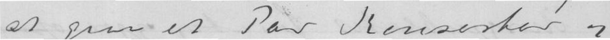at give et Par Konserter og
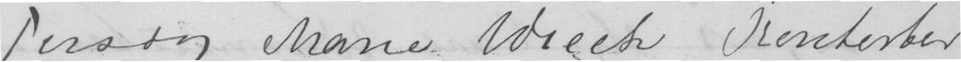Tirsdag Marie Wieck Konterter
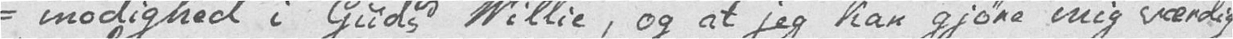-modighed i Guds Villie, og at jeg kan gjøre mig værdig
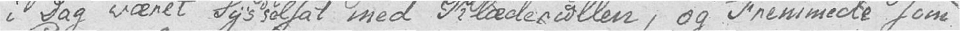i Dag været Sysselsat med Klæderullen, og Fremmede som
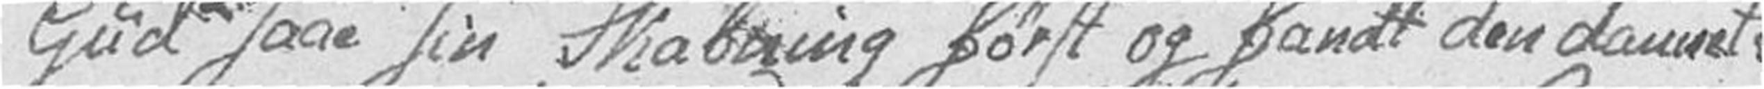Gud saae sin Skabning først og fandt den dannet
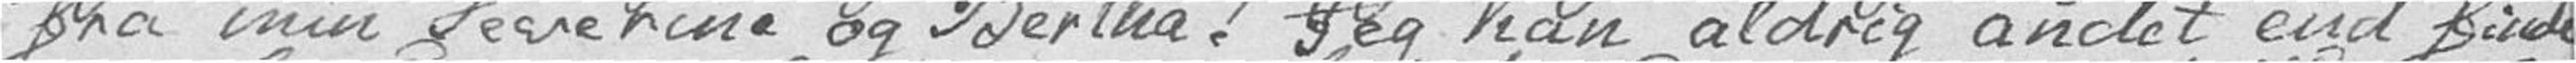fra min Severine og Bertha! Jeg kan aldrig andet end finde
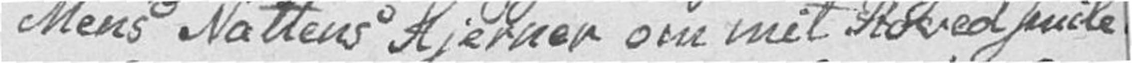Mens Nattens Stjerner om mit Hoved smile
